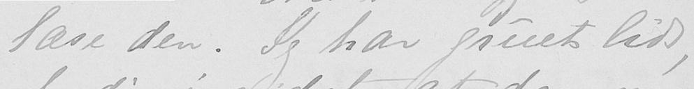læse den. Jeg har gruet lidt,
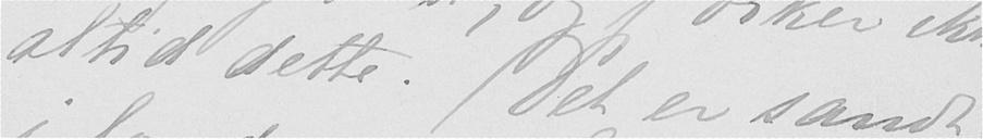altid dette. Det er sandt,
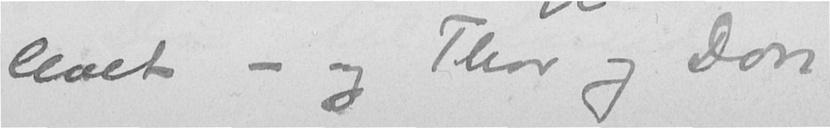levet - og Thor og Don
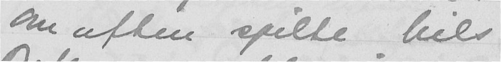Om aften spilte Nils
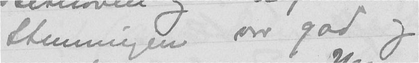Stemningen var god og
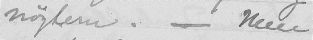nøgtern. - Men
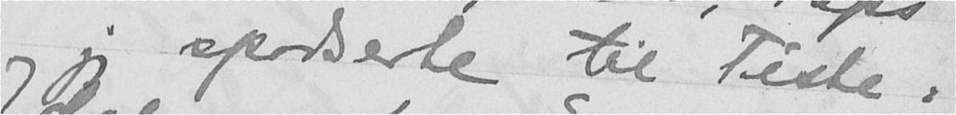og jeg spadserte til Tiste-
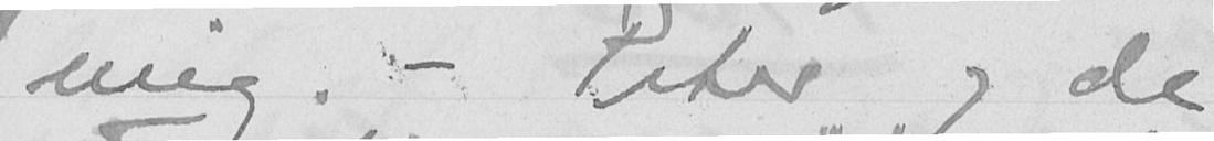mig. - Peter og de
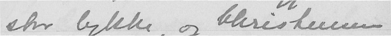stor lykke og Christensen
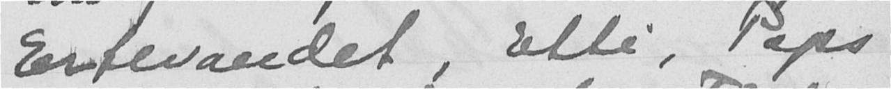Ertevandet, Etti, Paps
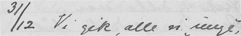31/12 Vi gik, alle vi "unge",
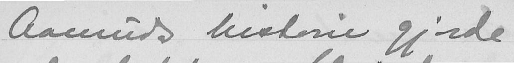Aanruds historie gjorde
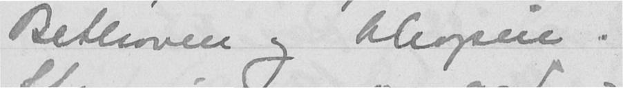Bethoven og Chopin.
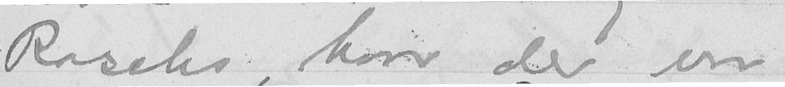Raschs, hvor der var
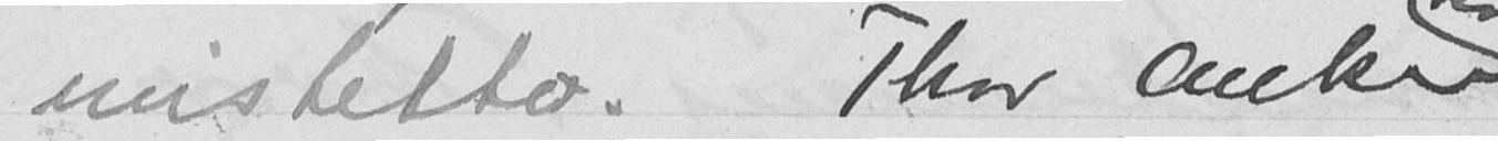mistelto. Thor Anker
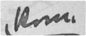kom
